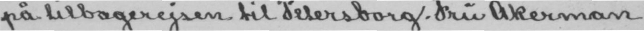på tilbagerejsen til Petersborg. Fru Åkerman
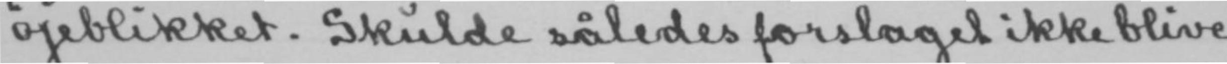øjeblikket. Skulde således forslaget ikke blive
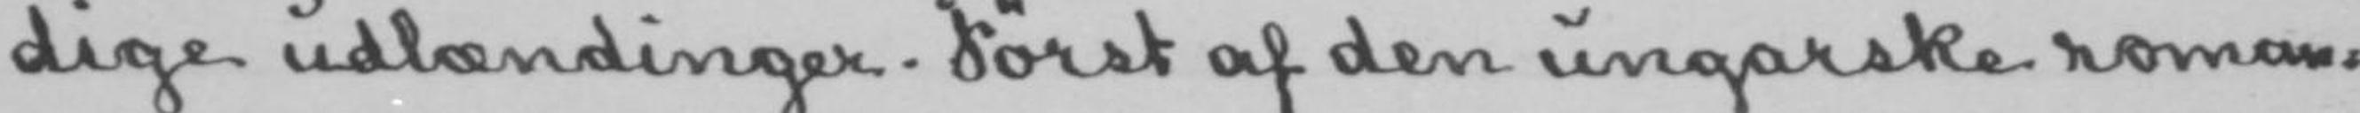dige udlændinger. Først af den ungarske roman-
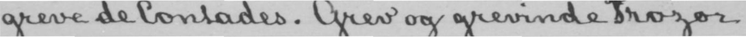greve de Contades. Grev og grevinde Prozor
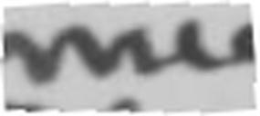mig
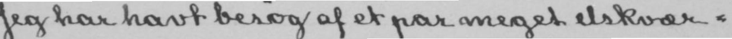Jeg har havt besøg af et par meget elskvær-
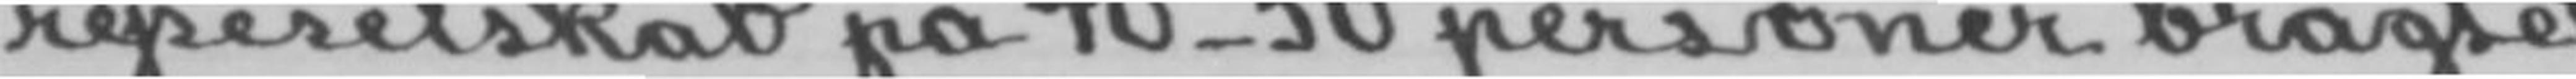rejseselskab på 40-50 personer bragte
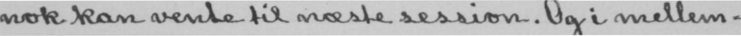nok kan vente til næste session. Og i mellem-
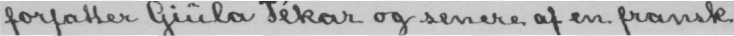forfatter Giula Pékar og senere af en fransk
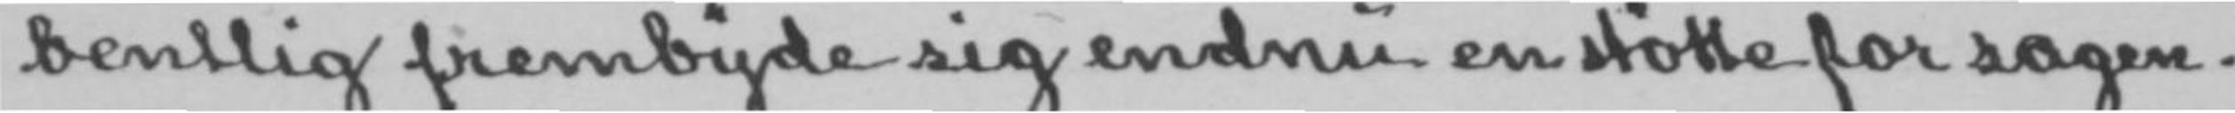bentlig frembyde sig endnu en støtte for sagen.
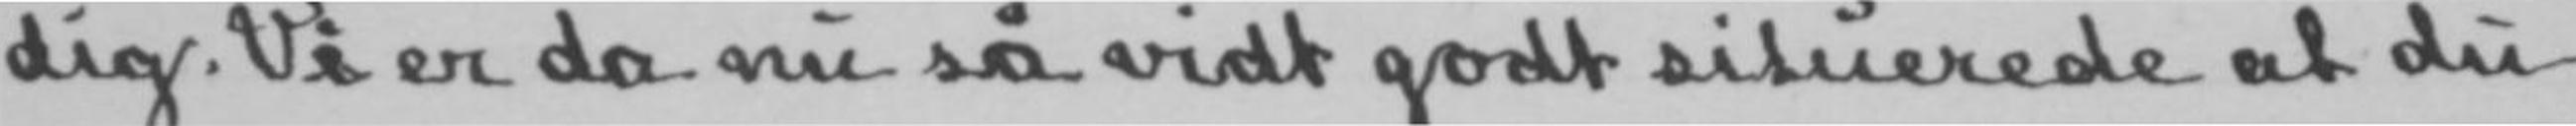dig. Vi er da nu så vidt godt situerede at du
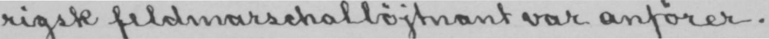rigsk feldmarschalløjtnant var anfører.
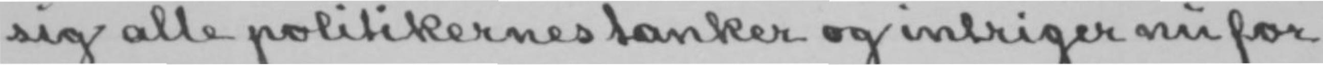sig alle politikernes tanker og intriger nu for
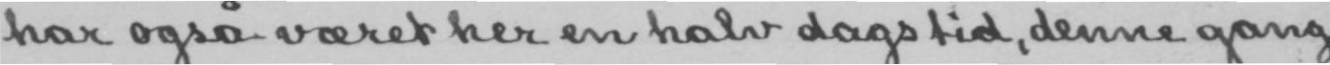har også været her en halv dags tid, denne gang
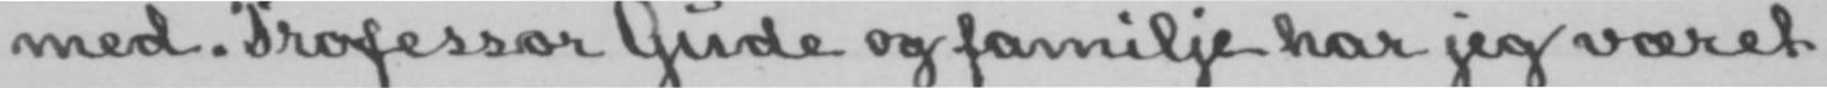med. Professor Gude og familje har jeg været
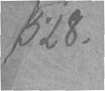328.
